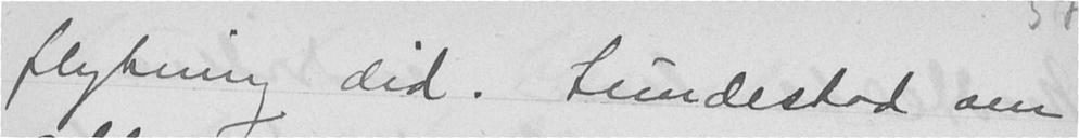flytning did. Lundestad om
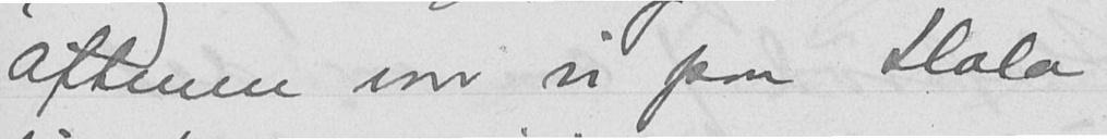aftenen var vi paa Hola
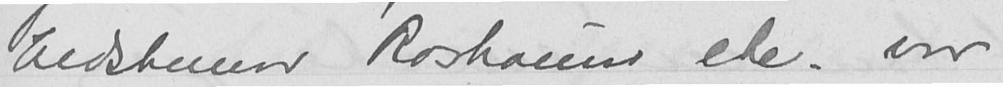bedstemor Roshauw etc. var
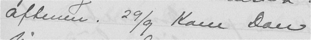aftenen. 29/9 Kom Don
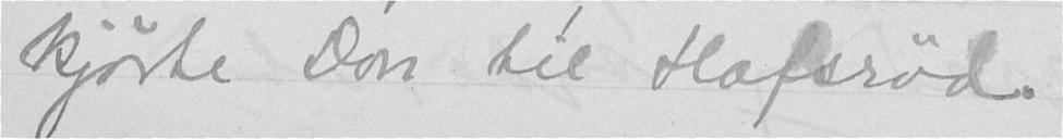kjørte Don til Hafsrød.
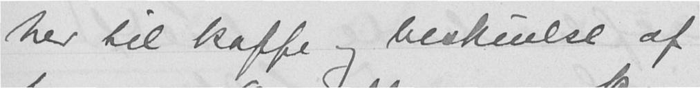her til kaffe og beskuelse af
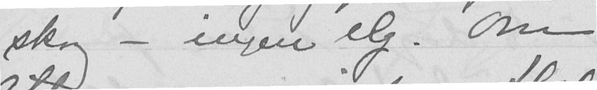skog - ingen elg. Om
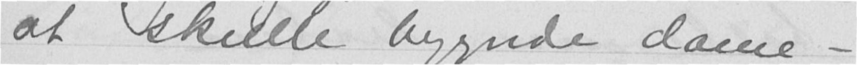at skulle begynde dame-
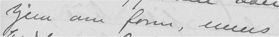hjem om form, mens
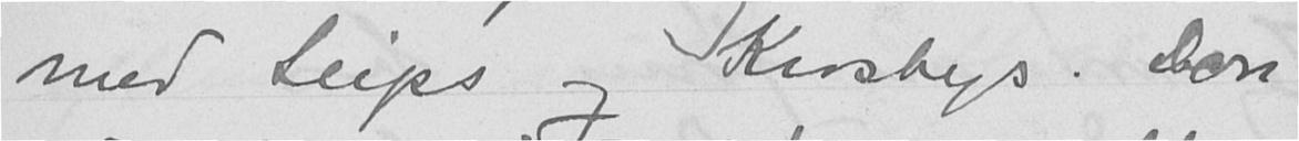med Seips og Krosbys. Don
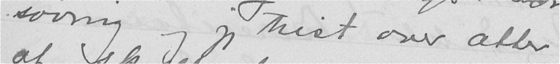søvnig og jeg trist over atter
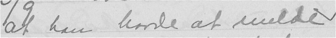at han havde at melde
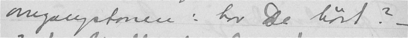omgangstonen: har De hørt? -
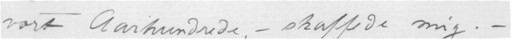vort Aarhundrede, - skaffede mig. -
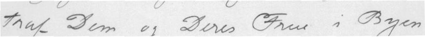traf Dem og Deres Frue i Byen
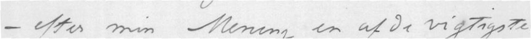- efter min Mening en af de vigtigste
